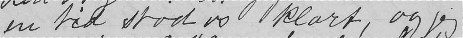en tid stod os klart, og jeg
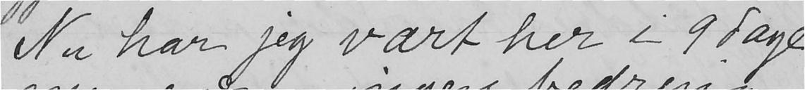Nu har jeg vært her i 9 dage
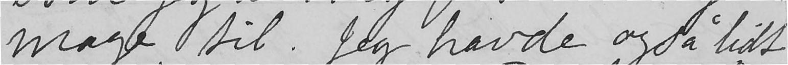mage til. Jeg havde også lidt
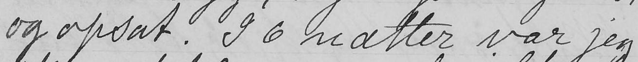og opsat. I 6 nætter var jeg
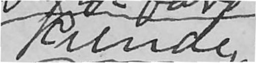kunde
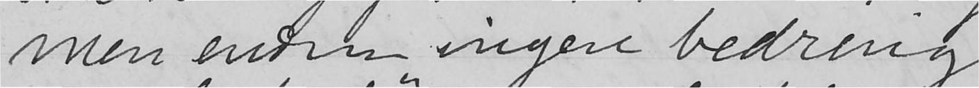men endnu ingen bedring
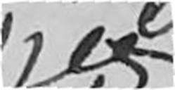jeg
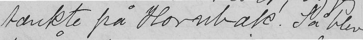tænkte på Hornbæk! Så blev
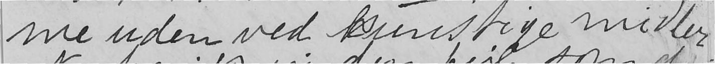me uden ved kunstige midler.
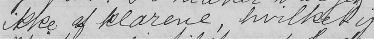ikke af klærene, hvilket ej
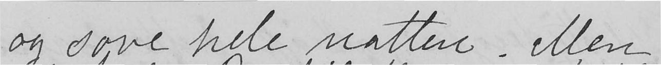og sove hele natten. Men
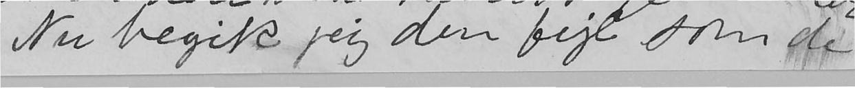Nu begik jeg den fejl som de
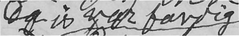Da jeg var færdig
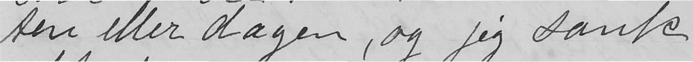ten eller dagen, og jeg sank
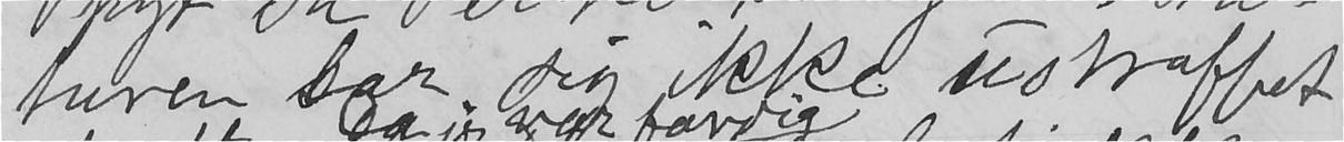turen lar sig ikke ustraffet
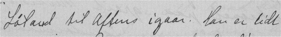Løland til aftens igaar. Han er lidt
